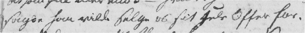sagde han vilde selge os sit hele Offer for.
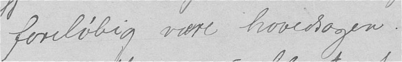foreløbig være hovedsagen.
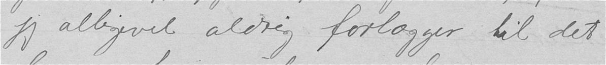jeg alligevel aldrig forlægger til det
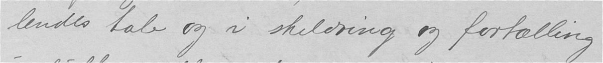lendes tale og i skildring og fortælling
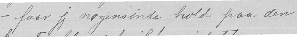- faar jeg nogensinde hold paa den
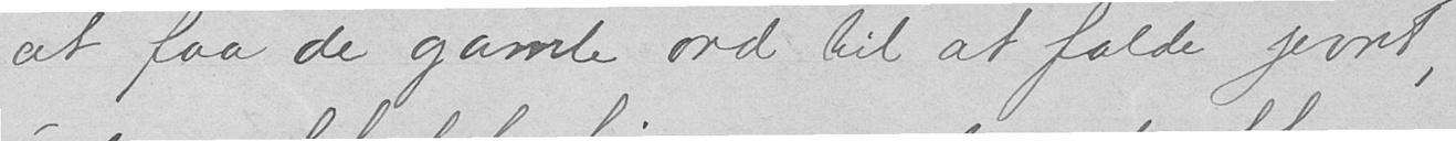at faa de gamle ord til at falde jevnt,
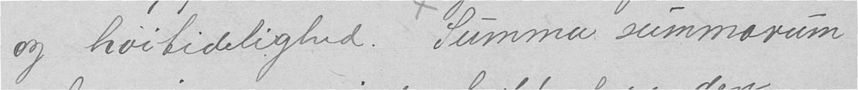og høitidelighed. Summa summarum
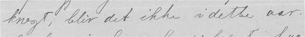knægt, blir det ikke i dette aar.
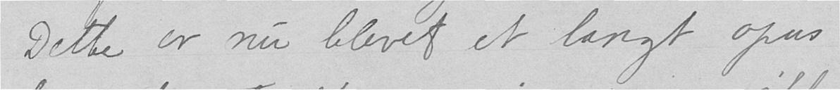Dette er nu blevet et langt opus
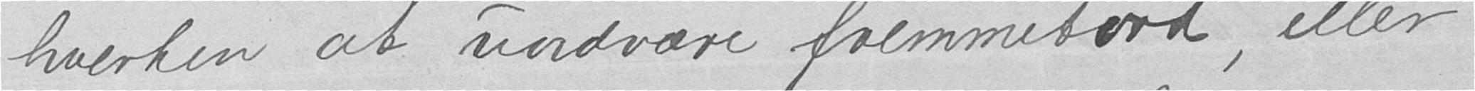hverken at undvære fremmedord, eller
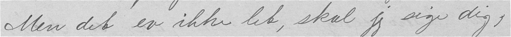Men det er ikke let, skal jeg sige dig,
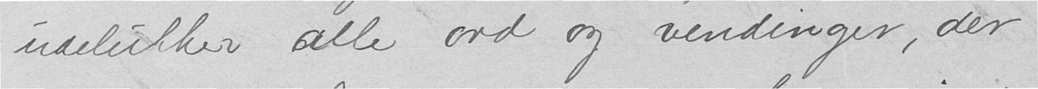udelukker alle ord og vendinger, der
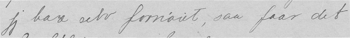jeg bare selv fornøiet, saa faar det
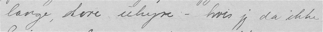lange, store uhyre - hvis jeg da ikke
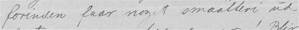forinden faar noget smaatteri ud
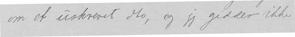om et uskrevet dito, og jeg gidder ikke
